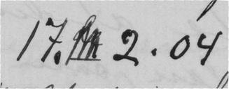17. 2.04
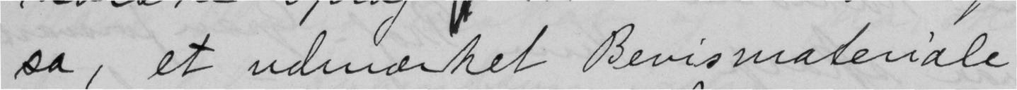sa, et udmærket Bevismateriale
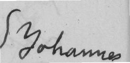Johannes
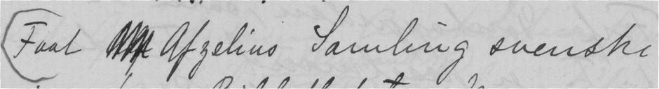Faat  Afzelius Samling svenske
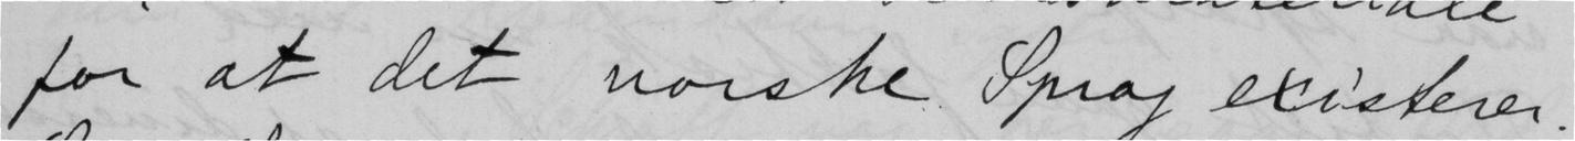for at det norske Sprog existerer.
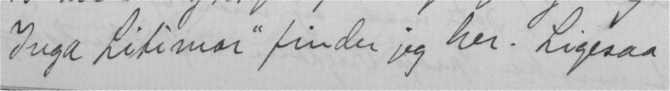Inga Litimar "finder jeg her. Ligesaa
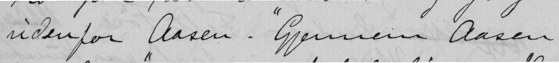udenfor Aasen. "Gjennem Aasen
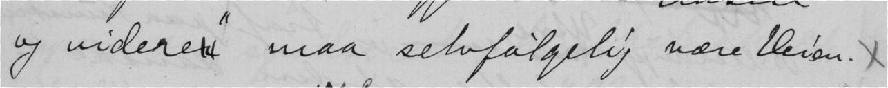og videre" maa selvfølgelig være veien. x
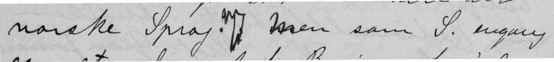norske Sprog. Men som S. engang
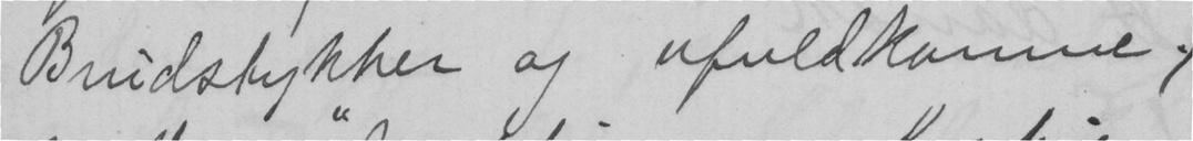Brudstykker og ufuldkomne.
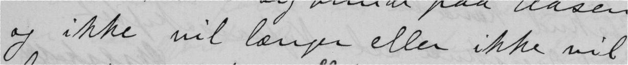og ikke vil længer eller ikke vil
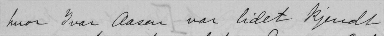hvor Ivar Aasen var lidet kjendt
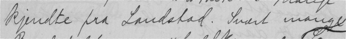kjendte fra Landstad. Svært mange
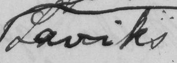Laviks
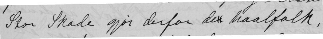Stor Skade gjør derfor de maalfolk,
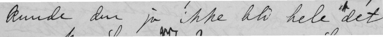kunde den jo ikke bli hele det
